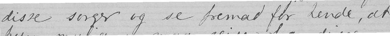disse sorger og se fremad for hende, at
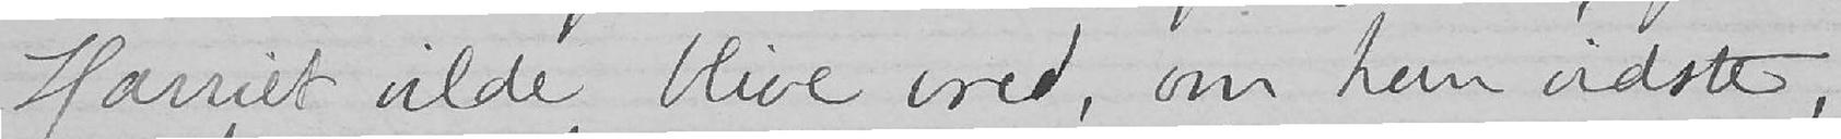Harriet vilde blive vred, om hun vidste,
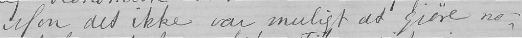Mon det ikke var muligt at gjøre no-
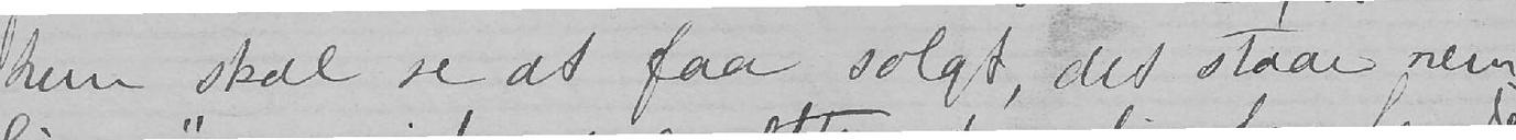hun skal se at faa solgt, det staar nem-
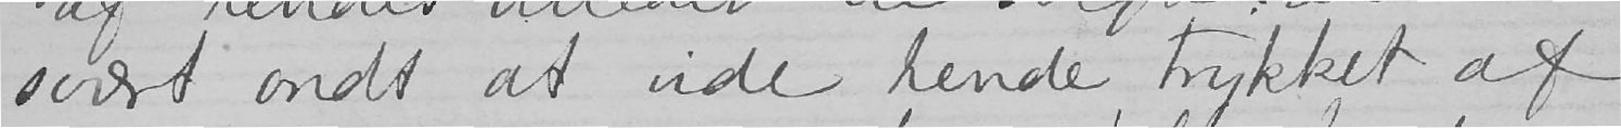svært ondt at vide hende trykket af
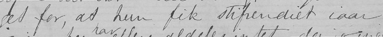get for, at hun fik stipendiet iaar
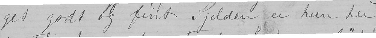get godt og fint. Sjelden er hun her
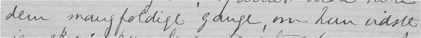dem mangfoldige gange, om hun vidste
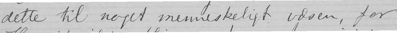dette til noget menneskeligt væsen, for
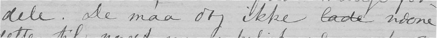dele. De maa dog ikke lade nævne
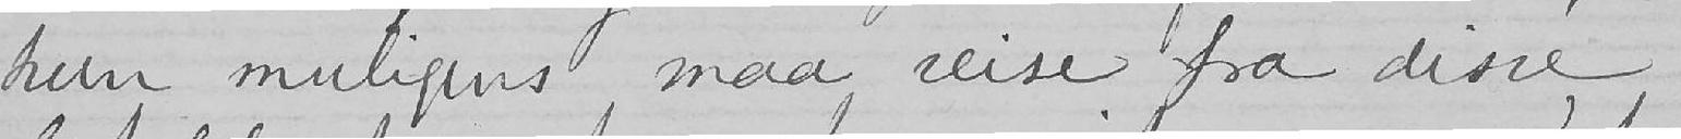hun muligens maa reise fra disse
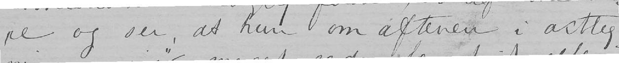re og ser, at hun om aftenen i actteg-
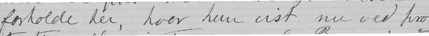forholde her, hvor hun vist nu ved pro
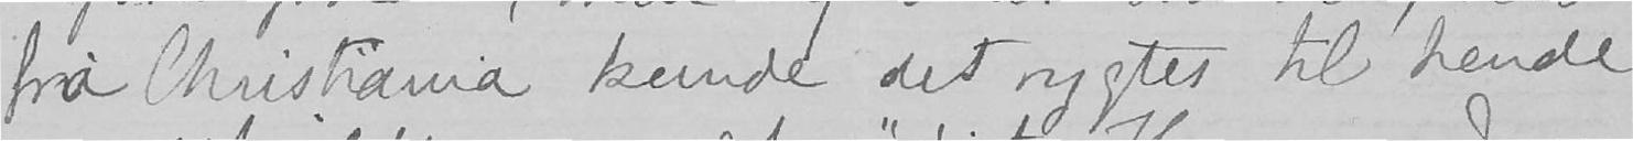fra Christiania kunde det rygtes til hende,
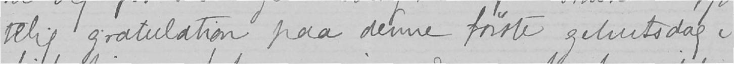telig gratulation paa denne første geburtsdag i
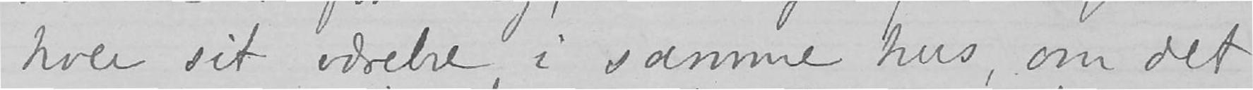hver sit værelse i samme hus, om det
